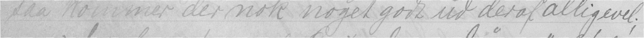saa kommer der nok noget godt ud deraf alligevel;
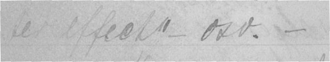ter effect" - osv. -
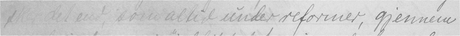sker det end, som altid under reformer, gjennem
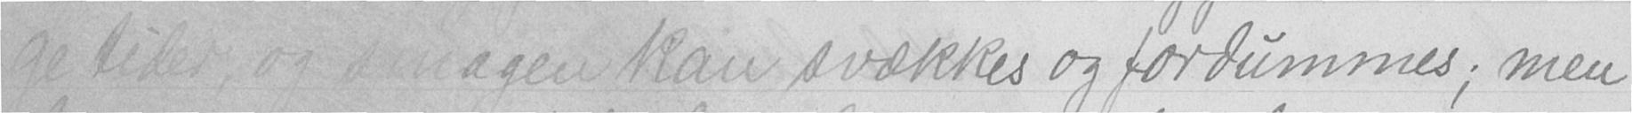ge tider, og smagen kan svækkes og fordummes; men
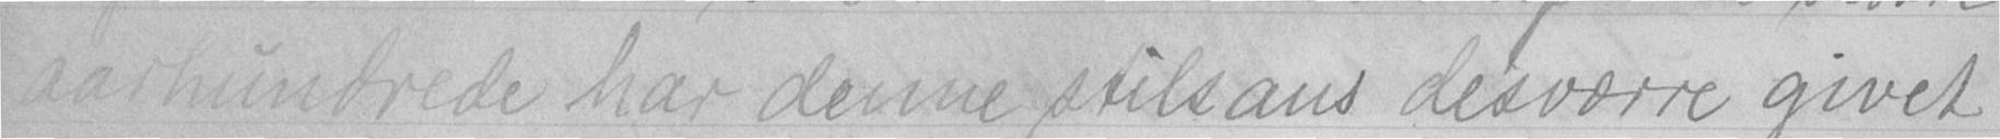aarhundrede har denne stilsans desværre givet
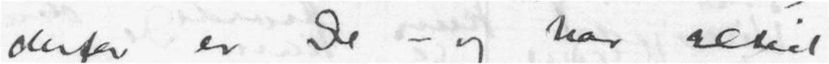derfor er De - og har altid
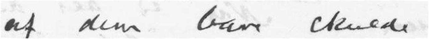af dem bare skulde
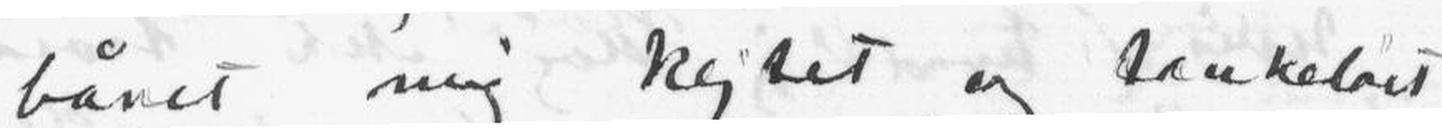båret mig kytet og tankeløst
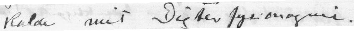kalde mit Digter fysionogmi.
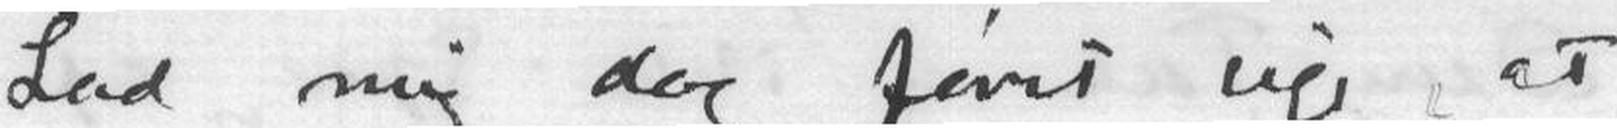Lad mig dog først sige at
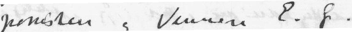ponisten og Vennen E. Gr.
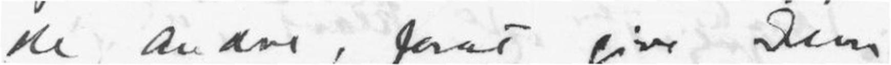de Andre, forat give Dem
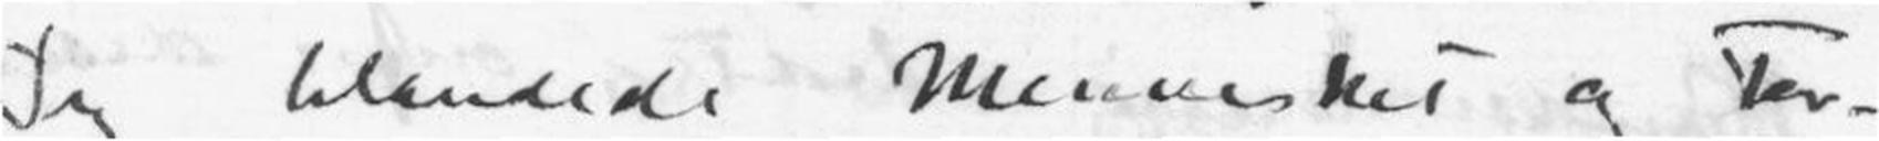Jeg blandede Mennesket og For-
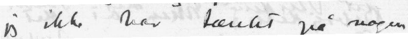jeg ikke har tænkt på nogen
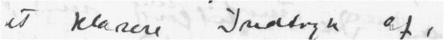et klarere Indtryk af,
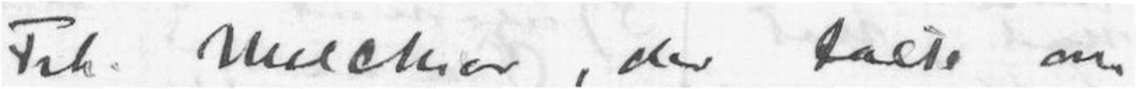Frk Melchior, der taste om
